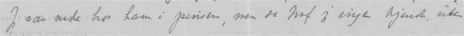Jeg var nede hos ham i pinsen, men da traf jeg ingen kjendte, uten
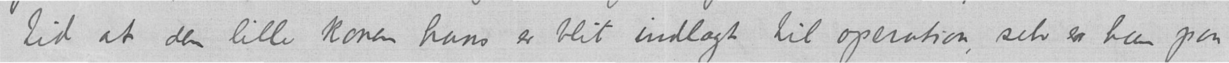tid at den lille konen hans er blit indlagt til operation, selv er han paa
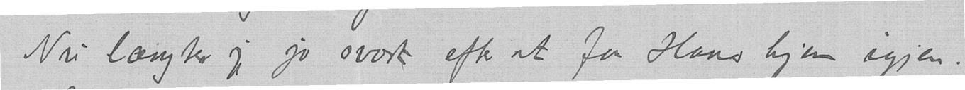Nu længter jeg jo svært efter at faa Hans hjem igjen.
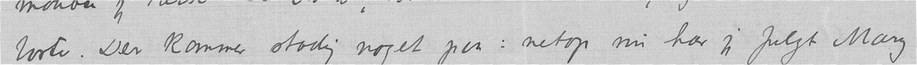borte. Der kommer stadig noget paa: netop nu har jeg fulgt Mary
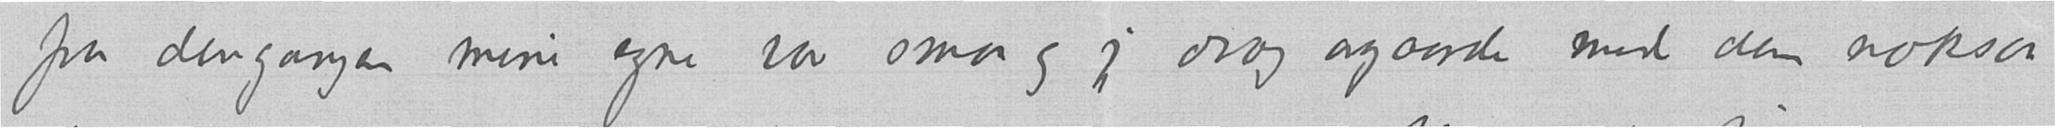fra dengangen mine egne var smaa og jeg drog avgaarde med dem noksaa
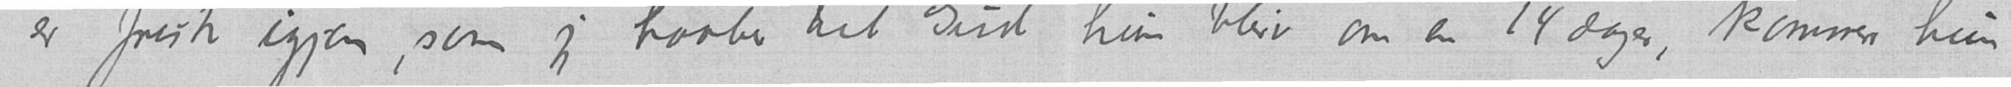er frisk igjen, som jeg haaber til Gud hun blir om en 14 dager, kommer hun
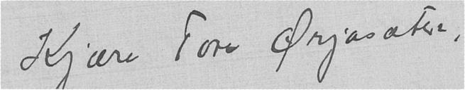Kjære Tore Ørjasæter
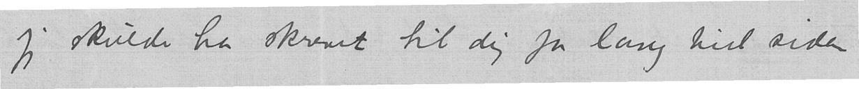jeg skulde ha skrevet til dig for lang tid siden
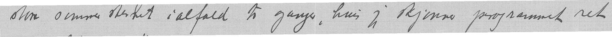store sommerstevnet ialfald to ganger, hvis jeg skjønner programmet ret
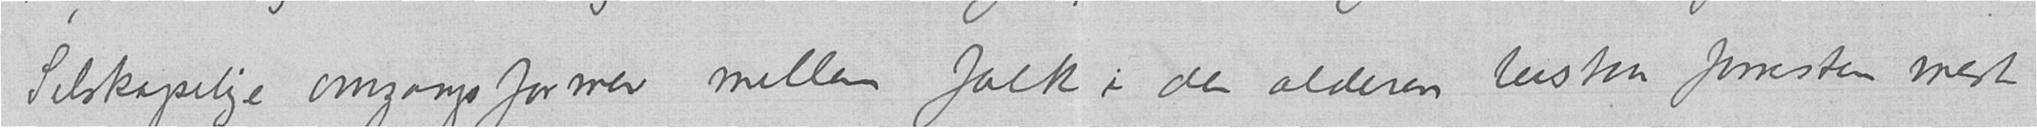Selskapelige omgangsformer mellem folk i den alderen bestaar forresten mest
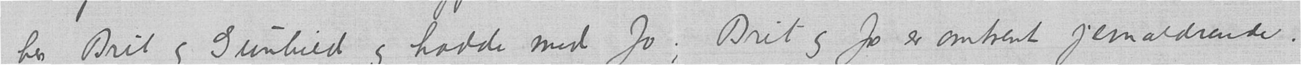her, Brit og Gunhild og hadde med Jo; Brit og Jo er omtrent jevnaldrende.
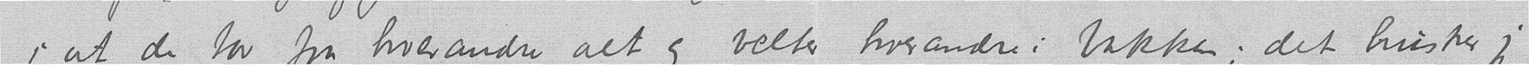i at de tar fra hverandre alt og velter hverandre i bakken; det husker jeg
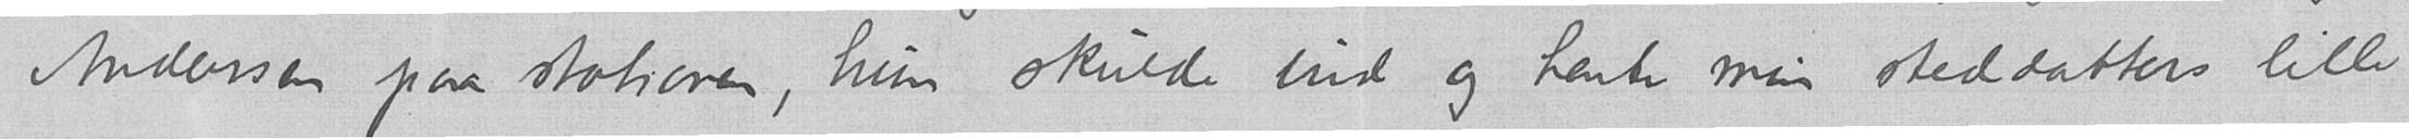Andersen paa stationen, hun skulde ind og hente min steddatters lille
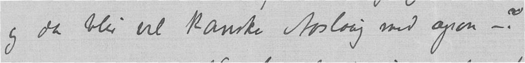og da blir vel kanske Aaslaug med ogsaa –?
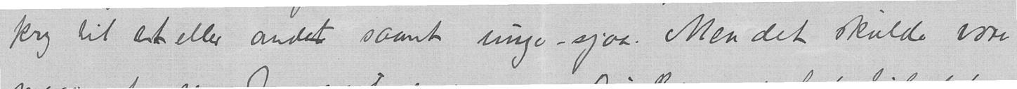kry til et eller andet saant unge-sjaa. Men det skulde være
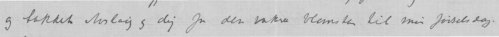og takket Aaslaug og dig for den vakre blomsten til min fødselsdag.
